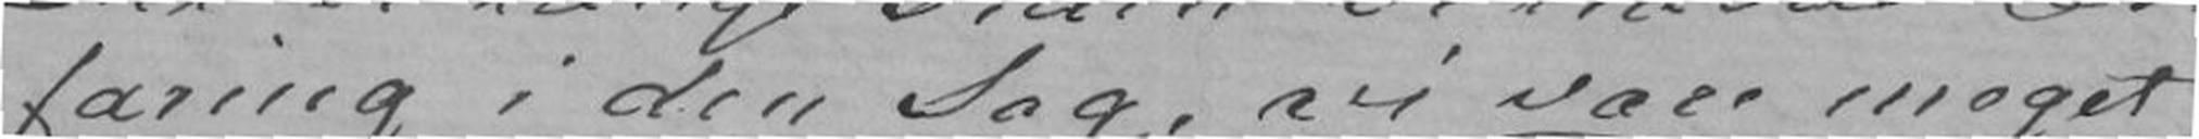faring i den Sag, vi være meget
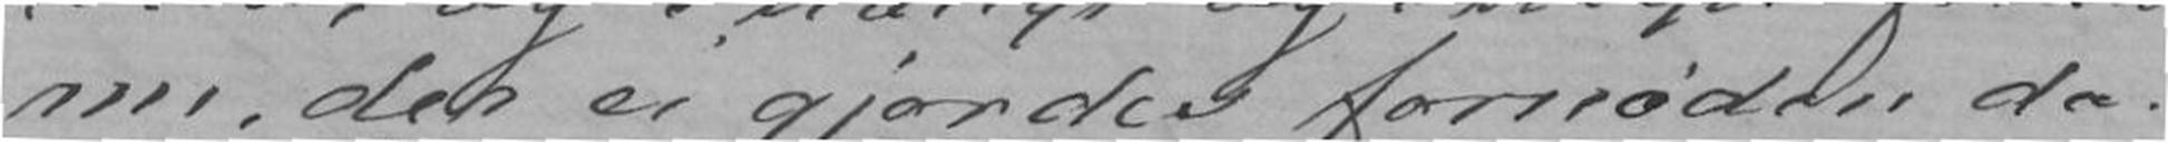nu, der ei gjordes fornøden da.
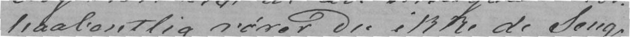haabentlig rører Du ikke de Seng-
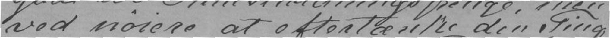ved nøiere at eftertænke den Ting
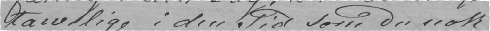tarvelige i den Tid som Du nok
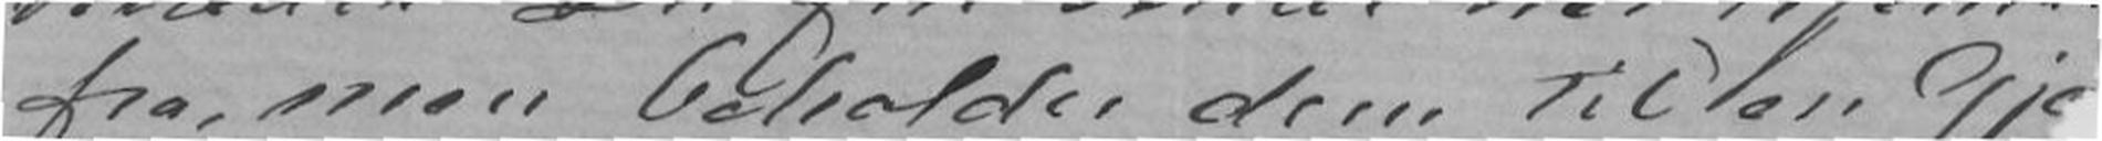fra, men beholder dem til en Gje
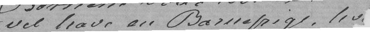vel have en Barnepige, hv
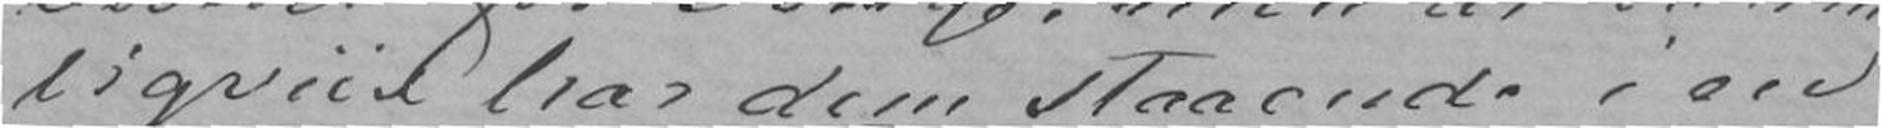ligviis har dem staaende i en
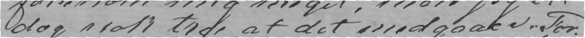dog nok troe at det medgaaer. For-
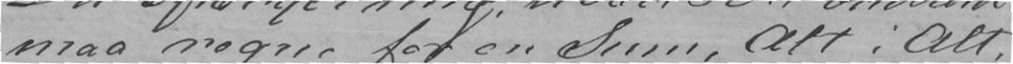maa regne for en Sum, Alt i Alt,
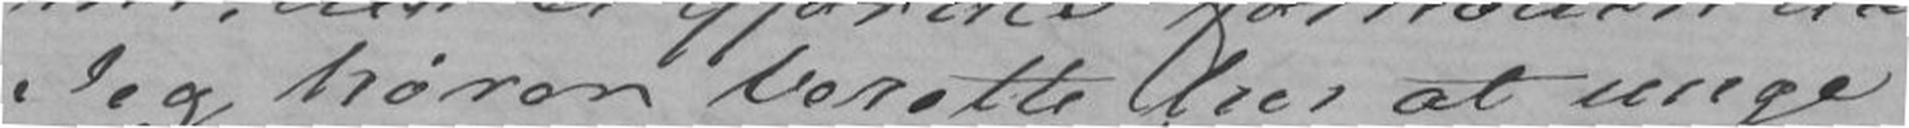Jeg hører berette her at unge
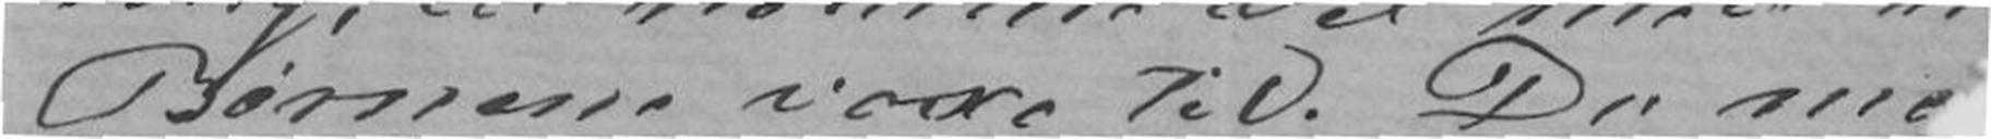Børnene voxe til Du me
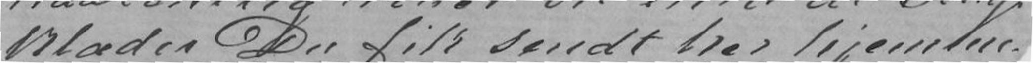klæder Du fik sendt her hjemme-
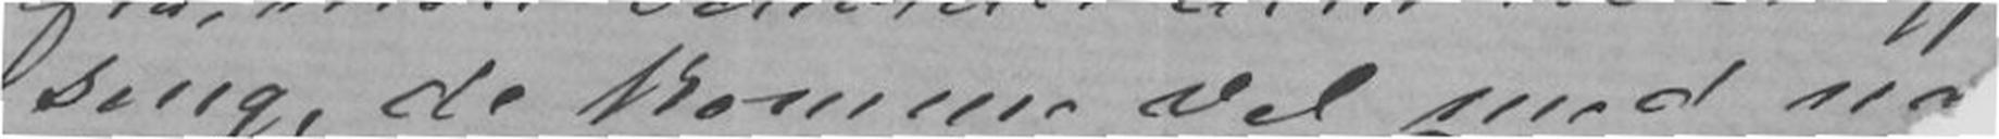seng, de komme vel med na
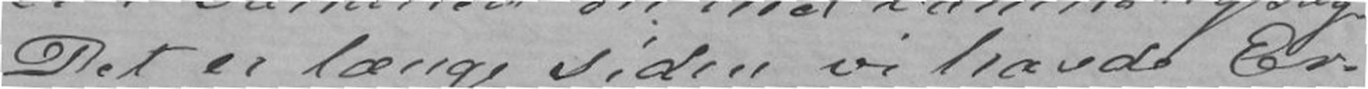Det er længe siden vi havde Er-
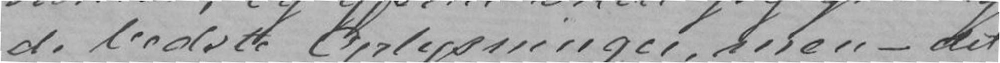de bedste Oplysninger, men - det
